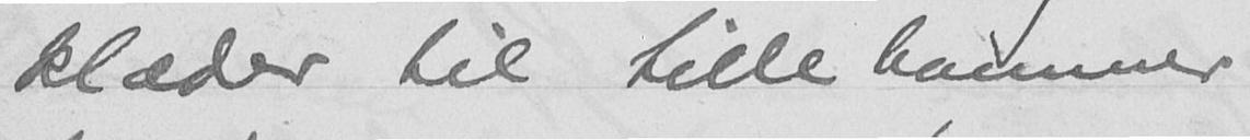klæder til Lillehammer
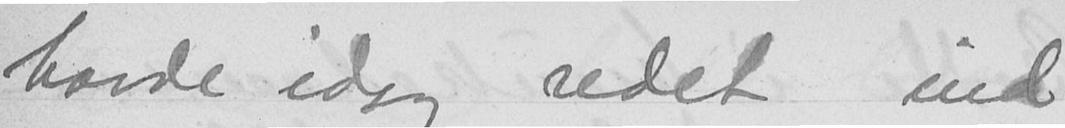havde idag redet ind
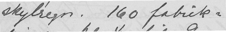skylregn. 160 fabrik-
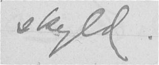skyld.
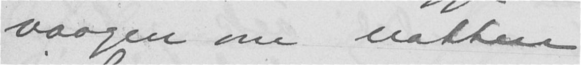vaagen om natten
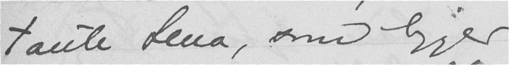Tante Lena, som ligger
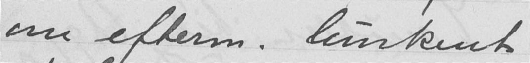om efterm. lunkent
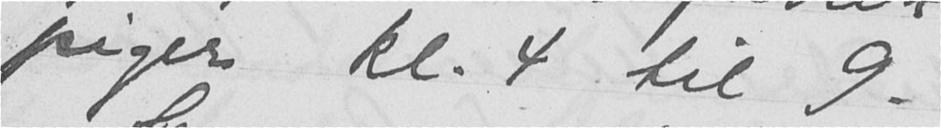piger kl. 4 til 9.
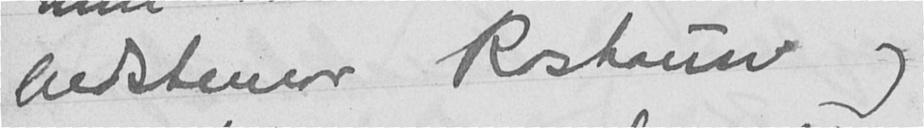bedstemor Roshauw og
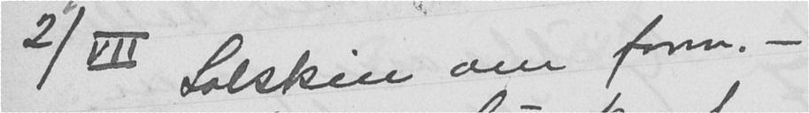2/VII Solskin om form. -
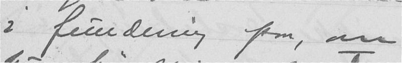i fundering paa, om
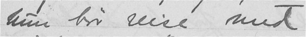hun bør reise med
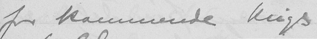for kommende krigs
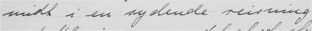midt i en sydende reisning
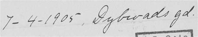7-4-1905, Dybwads gd.
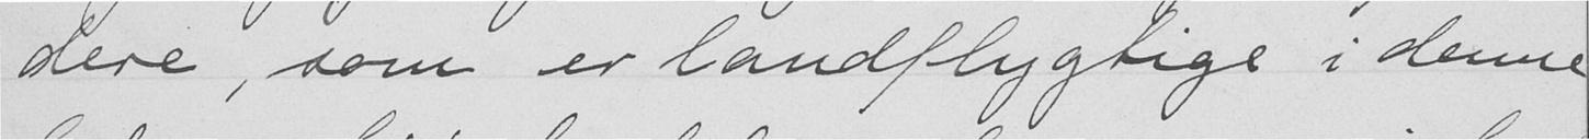dere, som er landflygtige i denne
